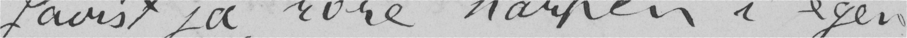Javist ja, røre harpen i egen-
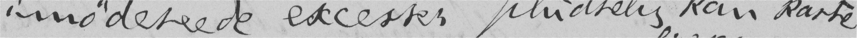imødeseede excesser pludselig kan kaste
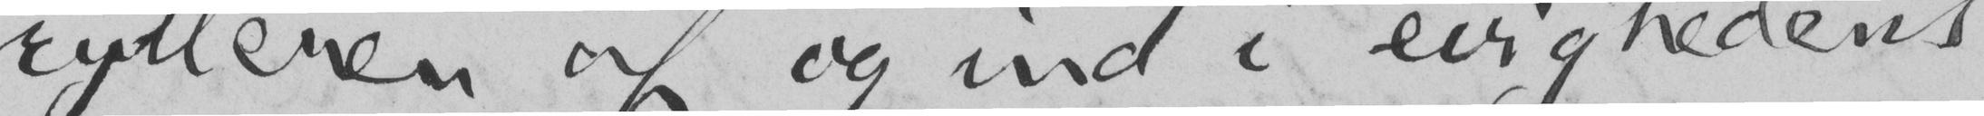rytteren af og ind i evighedens
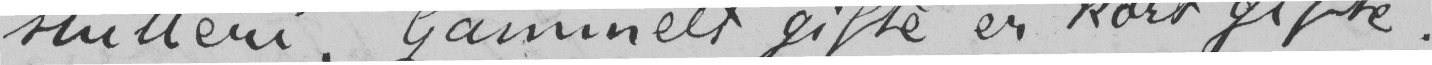stutteri. Gammelt gifte er kort gifte!
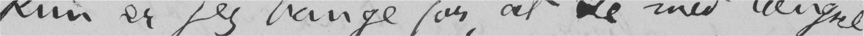Kun er jeg bange for, at de med længre
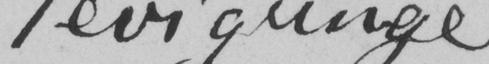evigunge
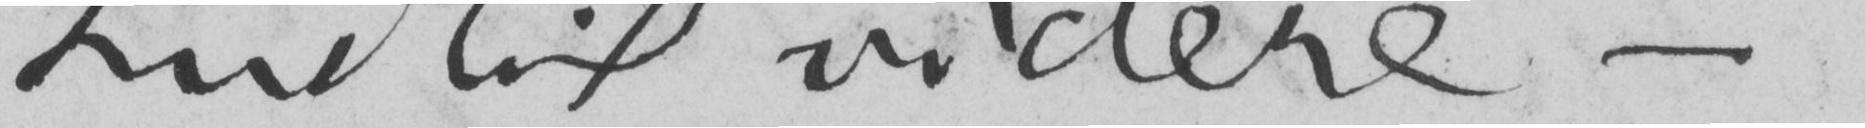Indtil videre –!
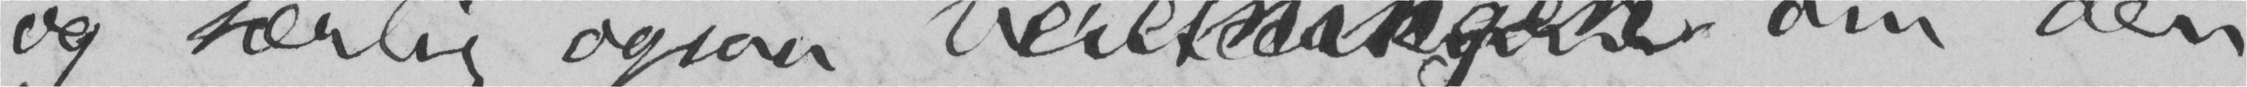og særlig ogsaa beretningen om den
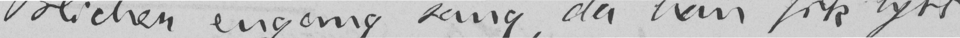Blicher engang sang, da han fik lyst
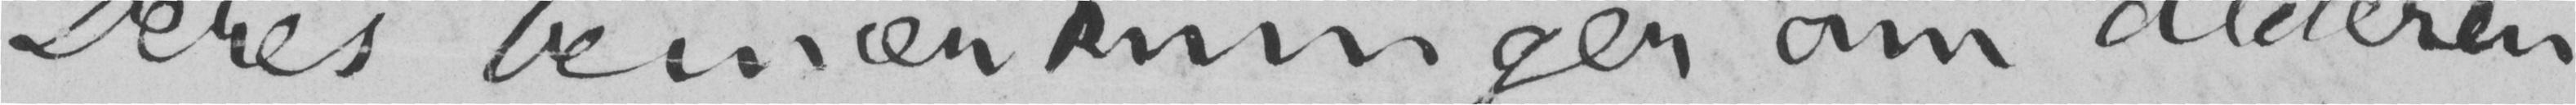Deres bemærkninger om alderen
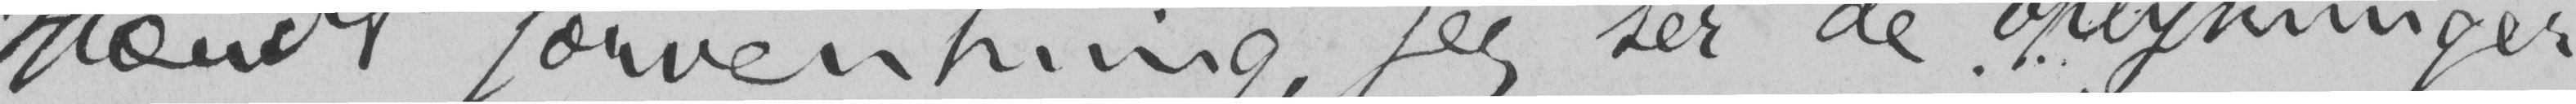spændt forventning, jeg ser de oplysninger
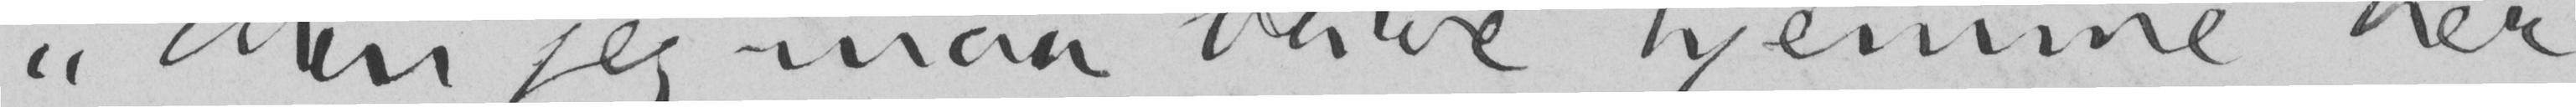«Men jeg maa blive hjemme her,
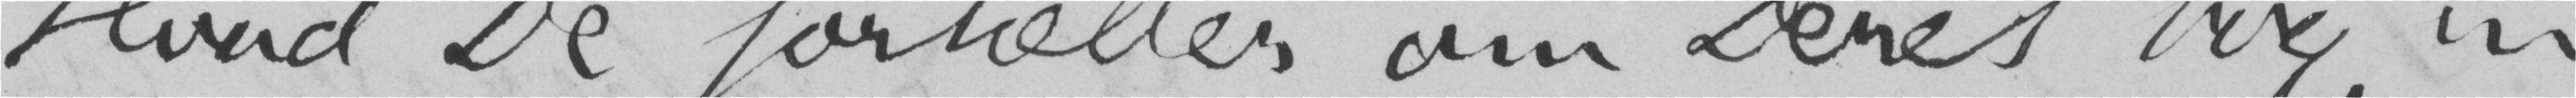Hvad De fortæller om Deres bog, in-
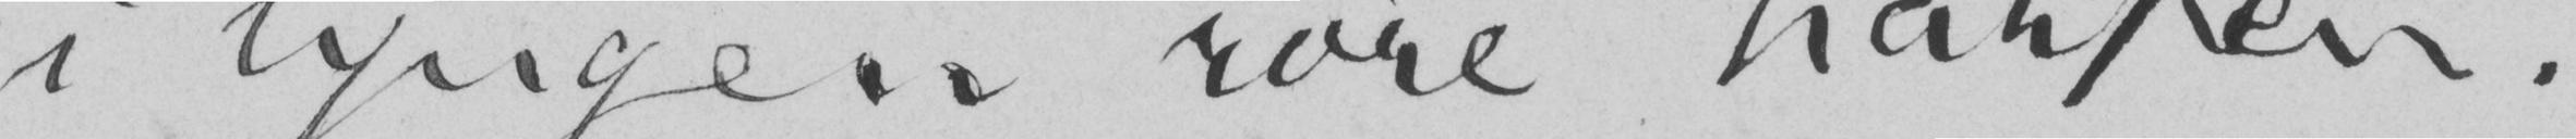i lyngen røre harpen.»
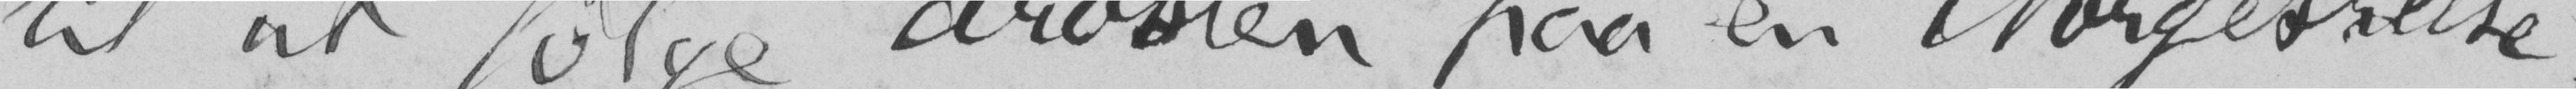til at følge droslen paa en Norgesreise:
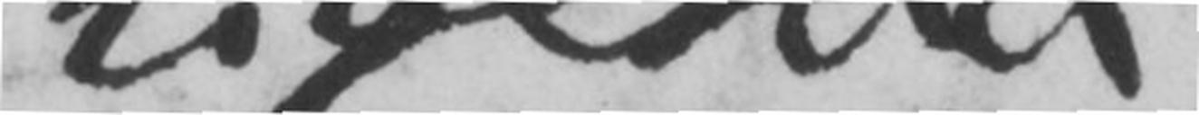ligesaa
